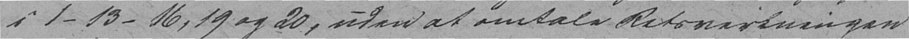i 1-13-16, 19 og 20, uden at omtale Retsvirkningen
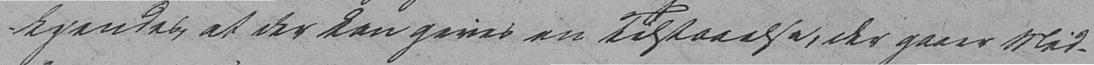kjendes, at der kan gives en Tilstaaelse, der gaaer Mid-
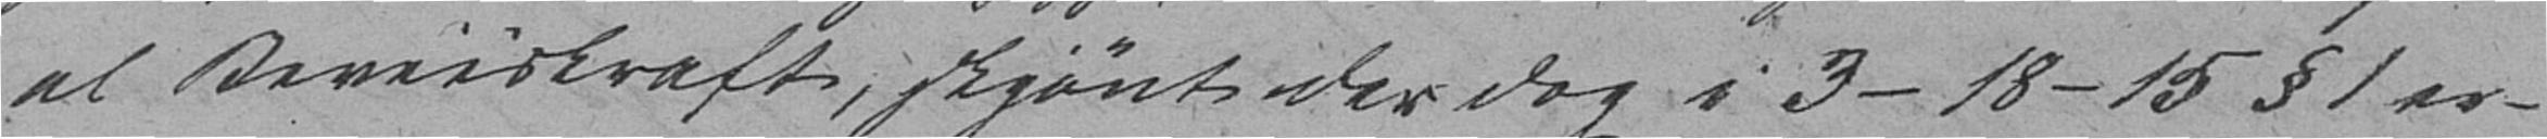al Beviiskraft, skjønt der dog i 3-18-15 § 1 er-
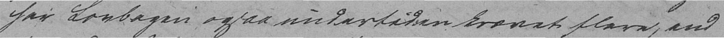har Lovbogen ogsaa undertiden krævet flere, end
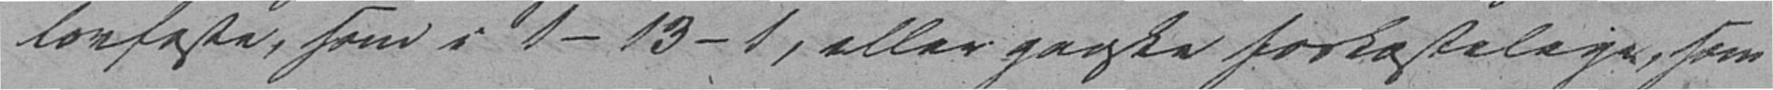lovfaste, som i 1-13-1, eller ganske forkastelige, som
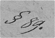§ 89.
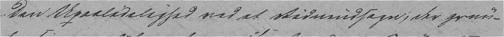Den Upaalidelighed ved et Vidneudsagn, der grun-
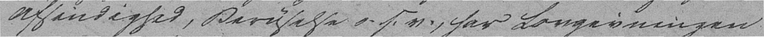Afsindighed, Beruselse o. s. v., har Lovgivningen
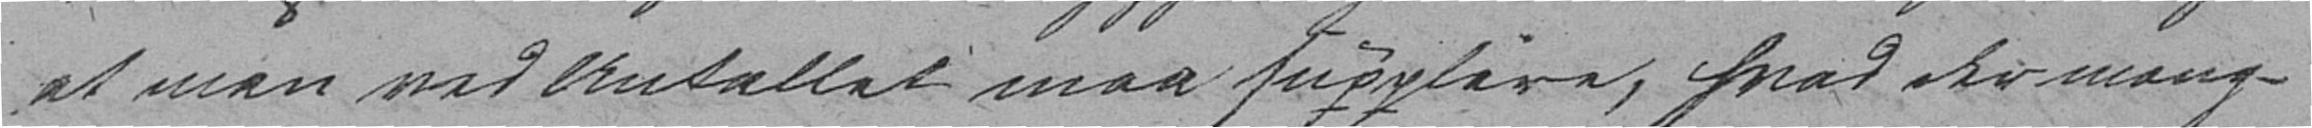at man ved Antallet maa supplere, hvad der mang-
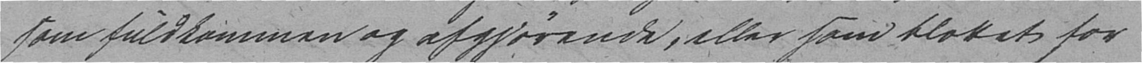som fuldkommen og afgjørende, eller som blottet for
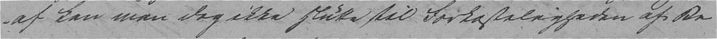af kan man dog ikke slutte til Forkasteligheden af Be-
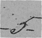- 5 -
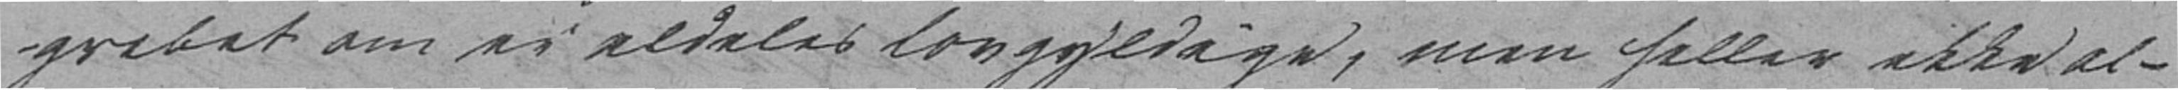grebet om ei aldeles lovgyldige, men heller ikke al-
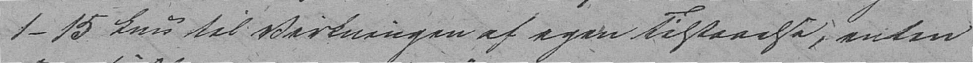1-15 kun til Virkningen af egen Tilstaaelse, enten
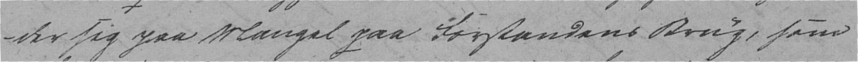der sig paa Mangel paa Forstandens Brug, som
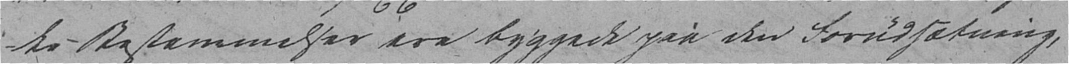ke Bestemmelser ere byggede paa den Forudsætning,
